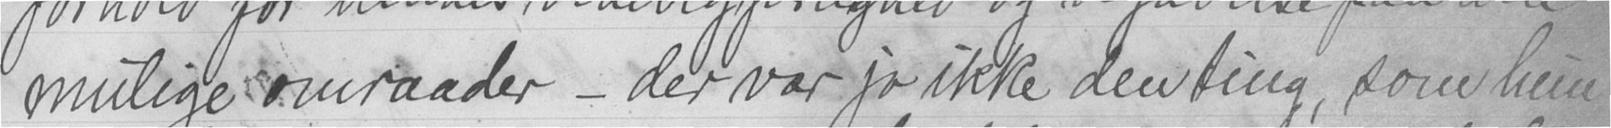mulige omraader - der var jo ikke den ting, som hun
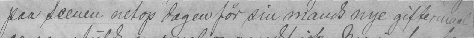paa scenen netop dagen før sin mands nye giftermaal
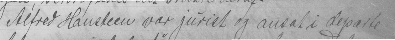Alfred Hansteen var jurist og ansat i departe-
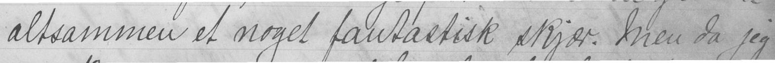altsammen et noget fantastisk skjær. Men da jeg
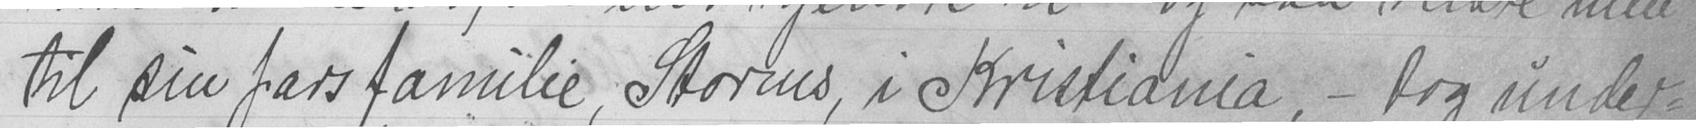til sin fars familie, Storms, i Kristiania, - tog under-
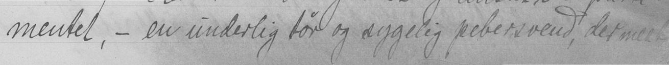mentet, - en underlig før og sygelig pebersvend, der mest
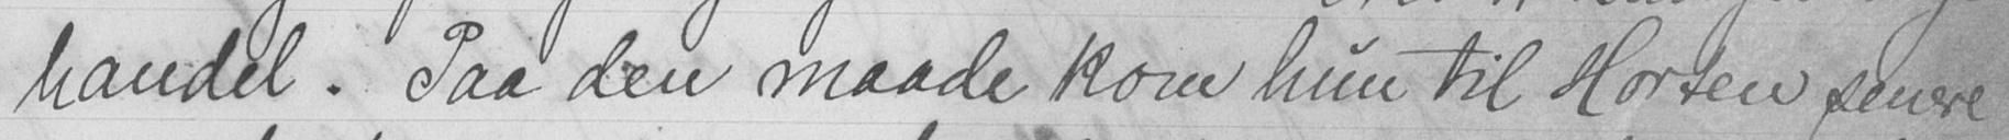handel. Paa den maade kom hun til Horten senere
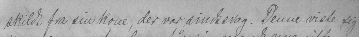skildt fra sin kone, der var sindssvag. Denne viste sig
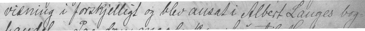visning i forskjelligt og blev ansat i Albert Langes bog-
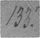133.
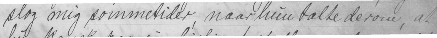slog mig sommetider, naar hun talte derom, at
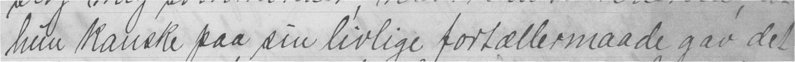hun kanske paa sin livlige fortællermaade gav det
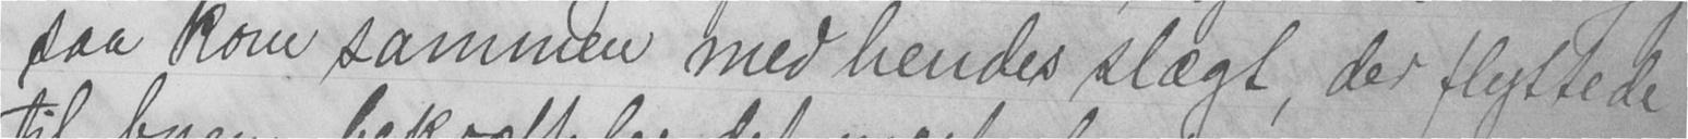saa kom sammen med hendes slægt, der flyttede
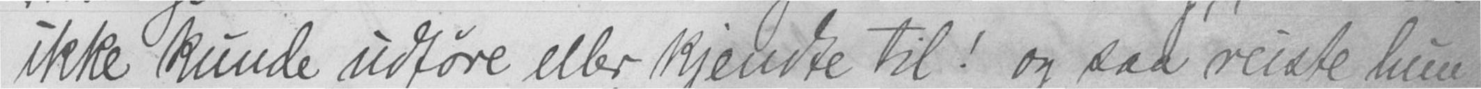ikke kunde udføre eller kjendte til! og saa reiste hun
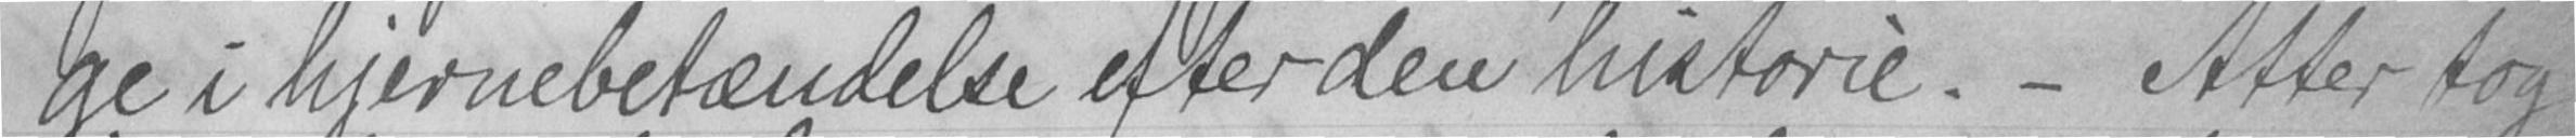ge i hjernebetændelse efter den historie. - After tog
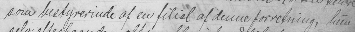som bestyrerinde af en filial af denne forretning, hun
